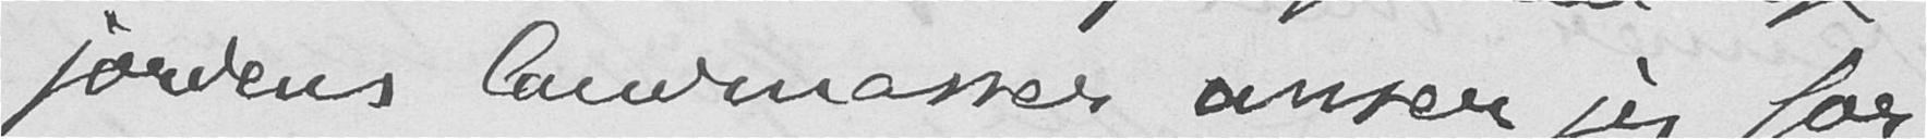jordens landmasser anser jeg for
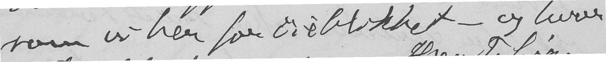som vi her for øieblikket - og hvor
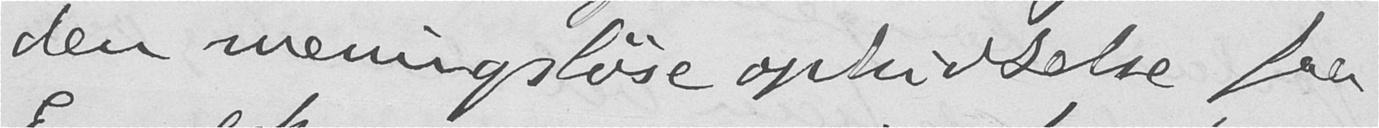den meningsløse ophidselse fra
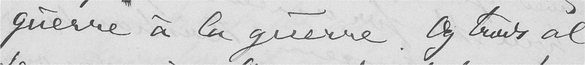guerre à la guerre. Og trods al
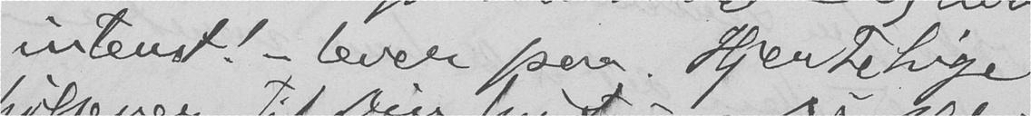intenst! - lever paa. Hjertelige
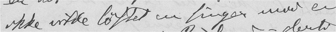ikke vilde løftet en finger mod en
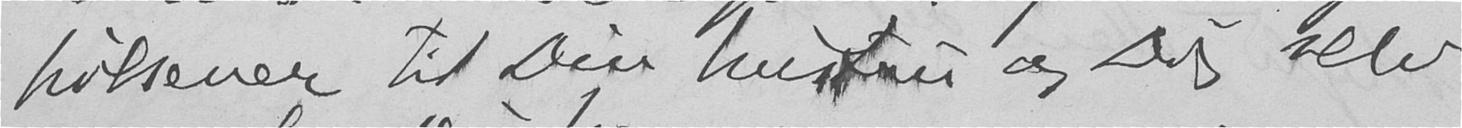hilsener til Din hustru og Dig selv
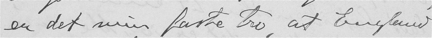er det min faste tro at England
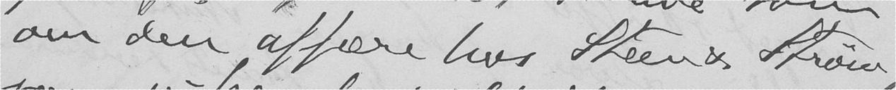om den affære hos Steen & Strøm,
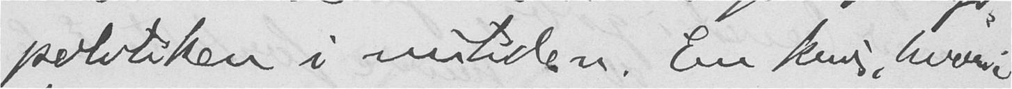politiken i nutiden. En krig, hvori
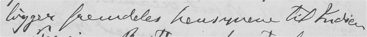ligger fremdeles hensynene til Indien
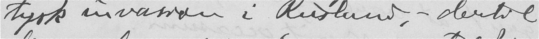tysk invasion i Rusland, - dertil
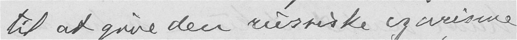til at give den russiske czarisme
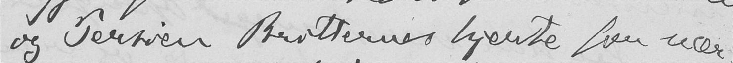og Persien Britternes hjerte for nær.
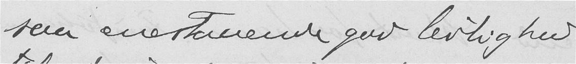saa enestaaende god leilighed
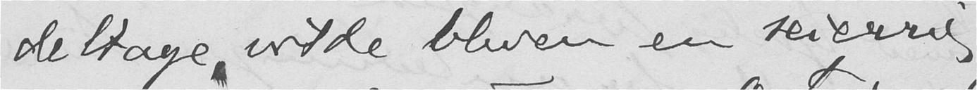deltage, vilde bleven en seierrig
